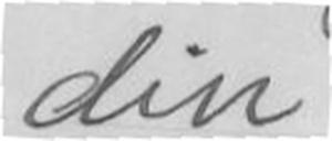din
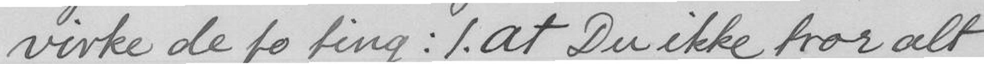virke de to ting: 1. At Du ikke tror alt
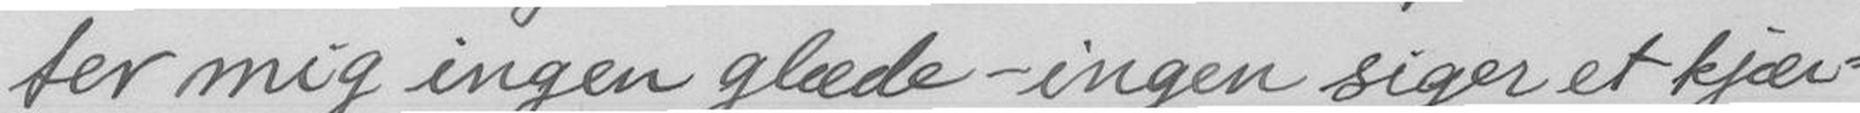ter mig ingen glæde - ingen siger et kjær-
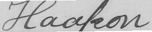Haakon.
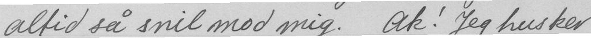altid så snild mod mig. Ak! Jeg husker
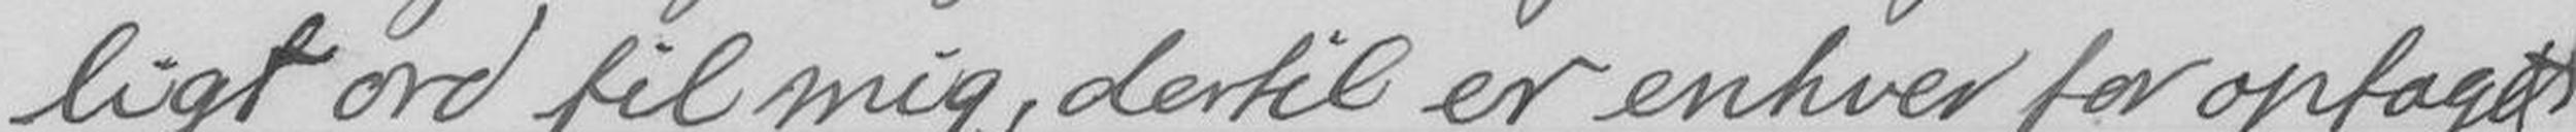ligt ord til mig, dertil er enhver for optaget
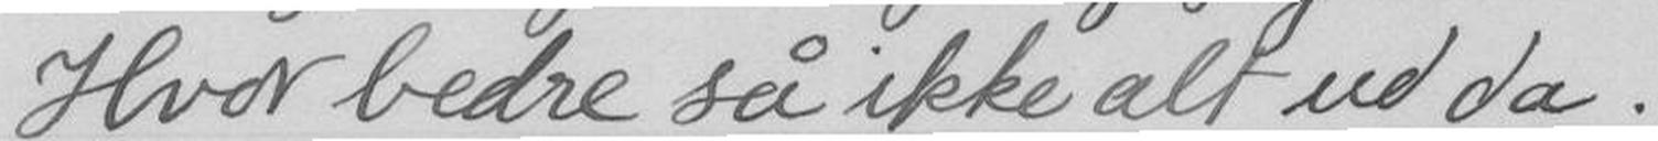Hvor bedre så ikke alt ud da.
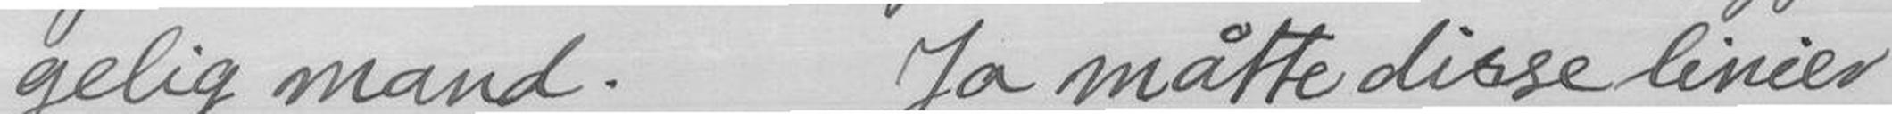gelig mand. Ja måtte disse linier
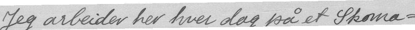Jeg arbeider her hver dag på et Skoma-
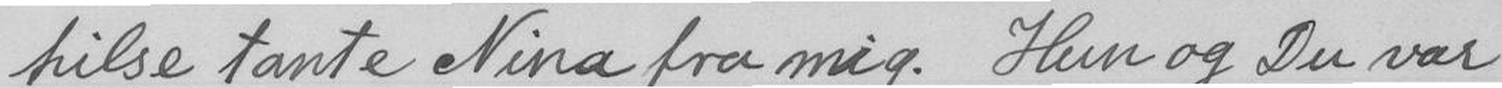hilse tante Nina fra mig. Hun og Du var
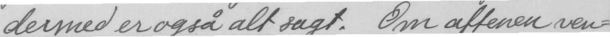dermed er også alt sagt. Om aftenen ven-
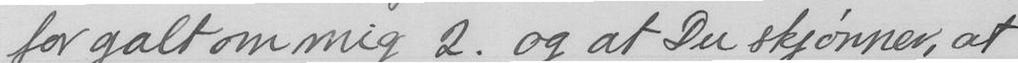for galt om mig 2. og at Du skjønner, at
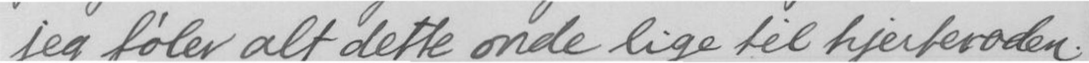jeg føler alt dette onde lige til hjerteroden.
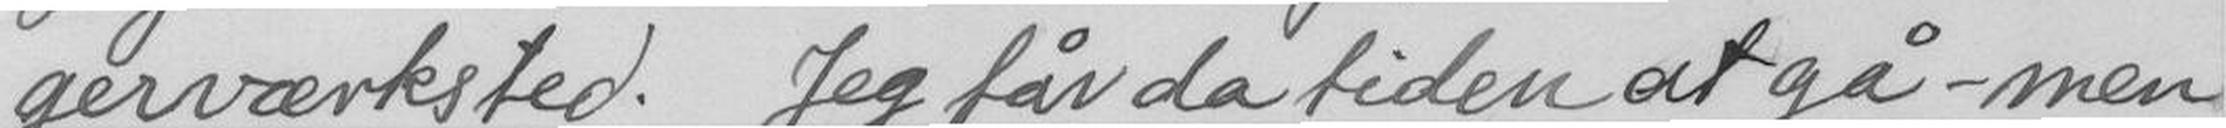kerværksted. Jeg får da tiden at gå - men
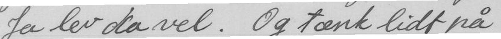Ja lev da vel. Og tænk lidt på
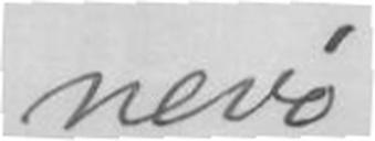nevø
